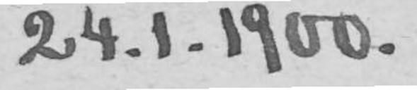24.1.1900.
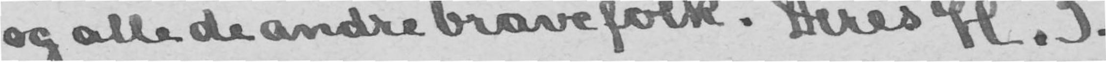og alle de andre brave folk. Deres H. I.
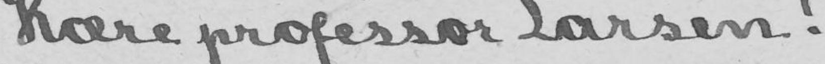Kære professor Larsen!
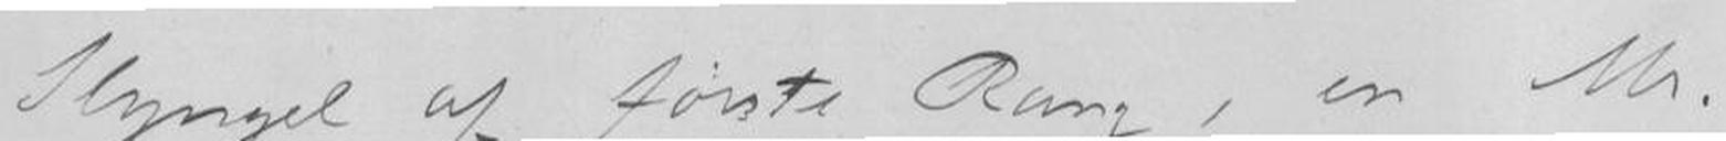Slyngel af første Rang, en Mr.
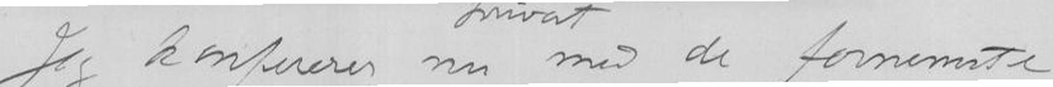Jeg konfererer nu med de fornemste
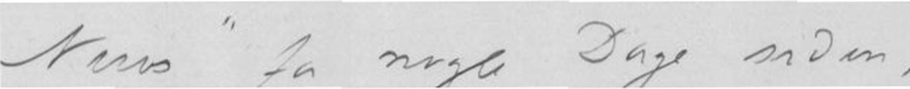News" for nogle Dage siden, i
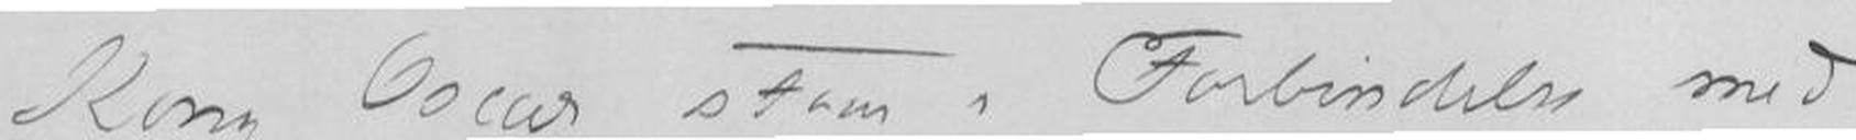Kong Oscar staar i Forbindelse med
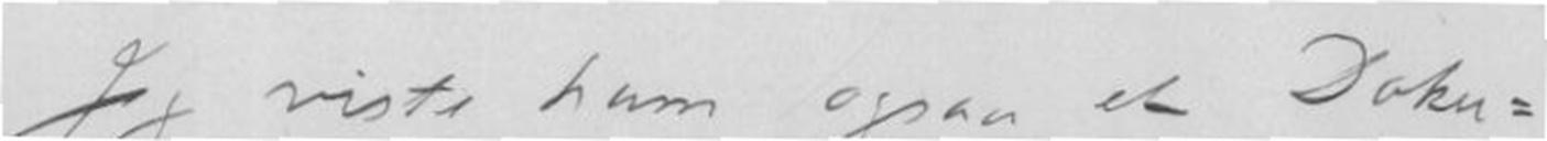Jeg viste ham ogsaa et Doku-
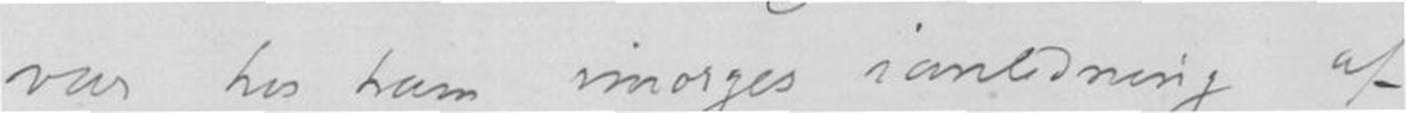var hos ham imorges ianledning af
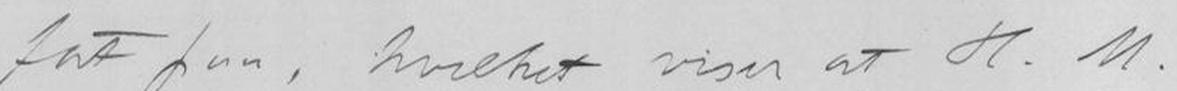fat paa, hvilket viser at H.M.
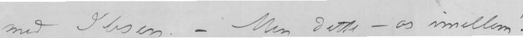med Ibsen. - Men dette - os imellem!
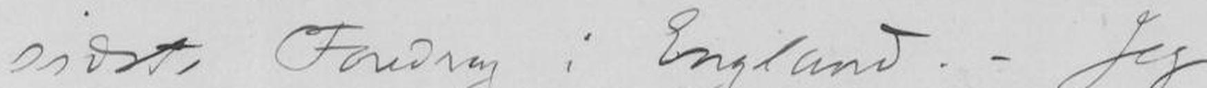sidste Foredrag i England. - Jeg
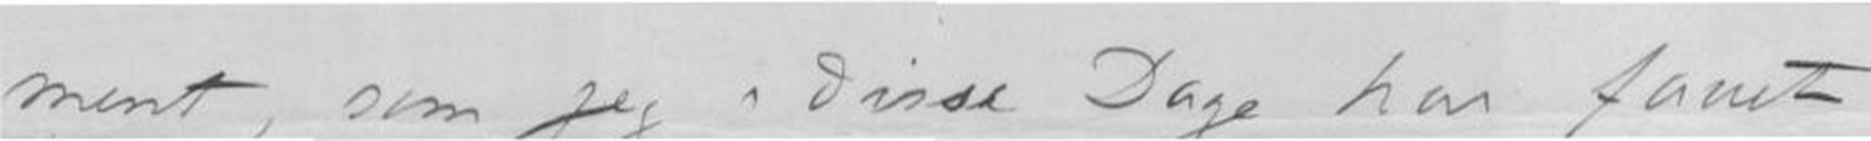ment, som jeg i disse Dage har faaet
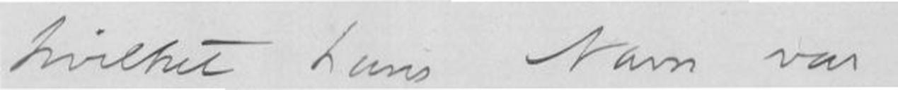hvilket hans Navn var
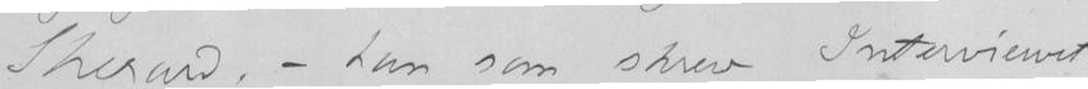Sherard, - han som skrev Interviewet
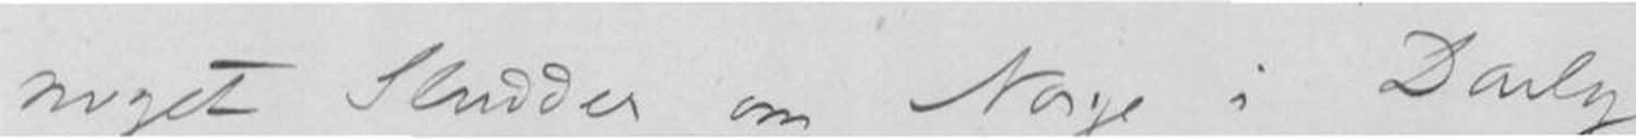noget Sludder om Norge i "Daily
